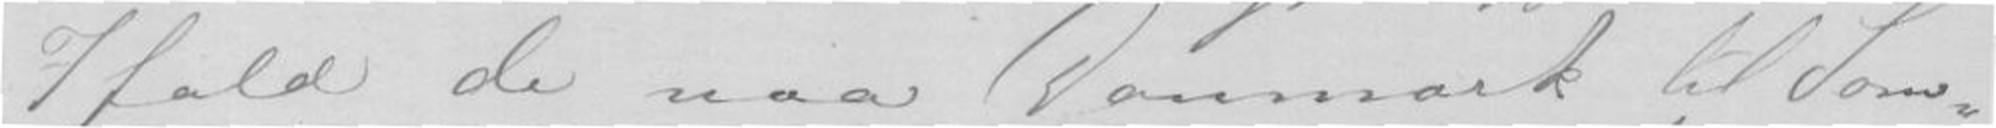I fald de naa Danmark til Som-
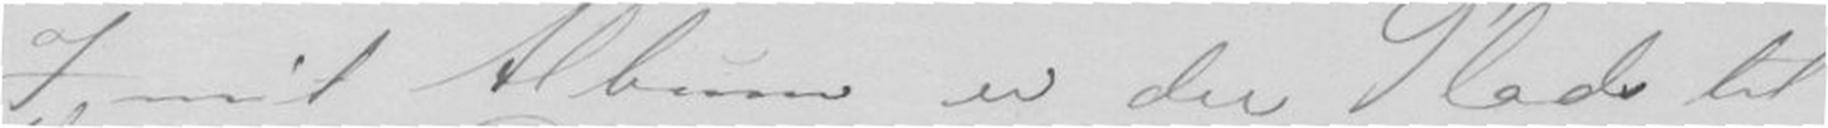I mit Album er der Plads til
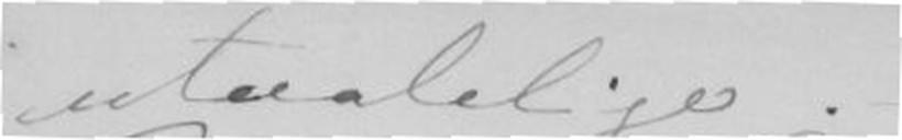utaalelige.
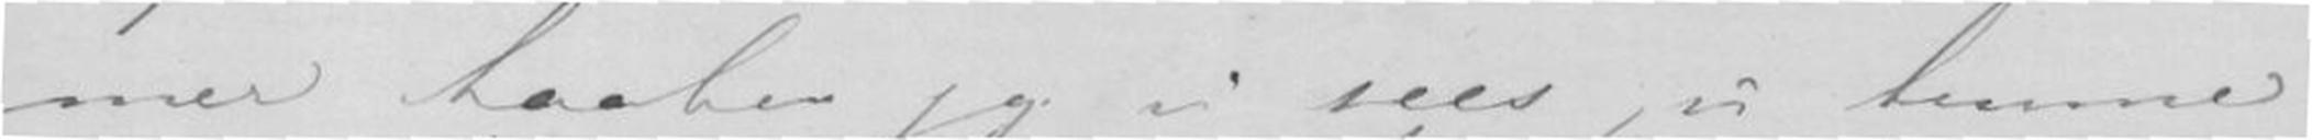mer haaber jeg vi sees, vi kunne
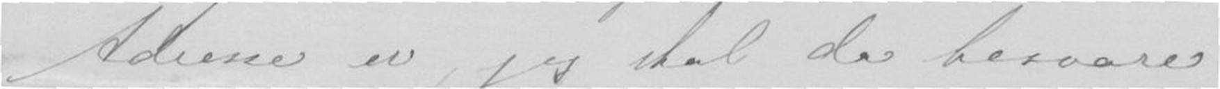Adresse er, jeg skal da besvare
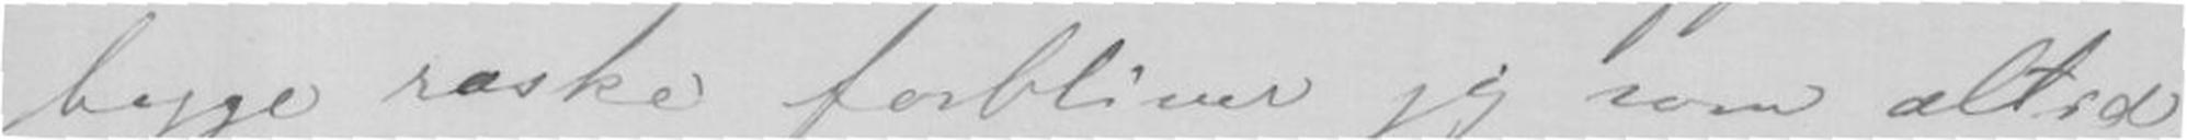begge raske forbliver jeg som altid
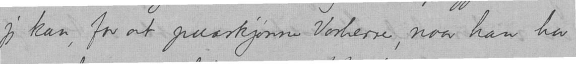jeg kan, for at paaskjønne Vorherre, naar han har
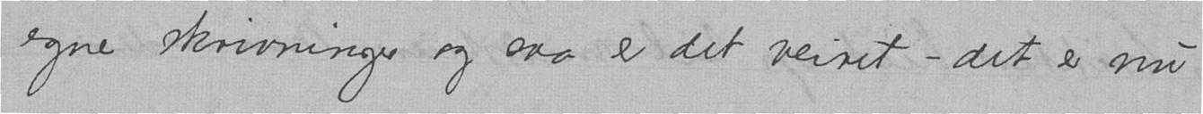egne skrivninger og saa er det veiret — det er nu
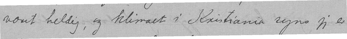været heldig, og klimaet i Kristiania syns jeg er
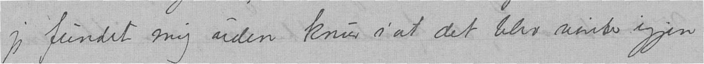jeg fundet mig uden knur i at det blir vinter igjen
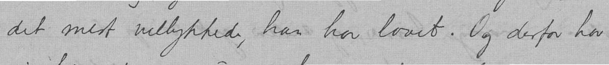det mest vellykkede, han har lovet. Og derfor har
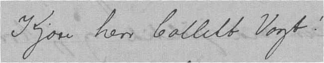Kjære herr Collett Vogt!
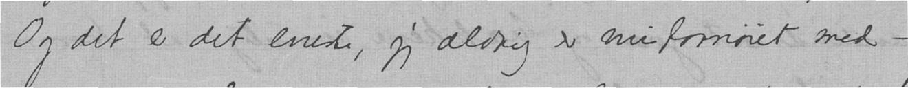Og det er det eneste, jeg aldrig er misfornøiet med -
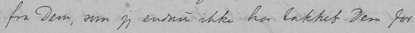fra Dem, som jeg endnu ikke har takket Dem for
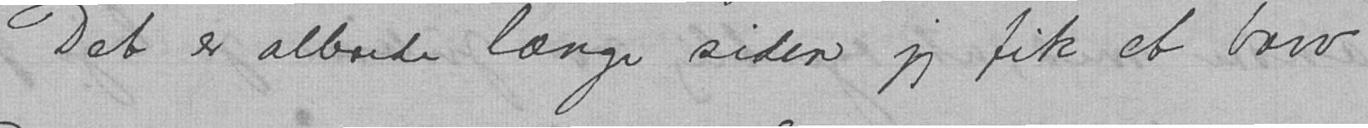Det er allerede længe siden jeg fik et brev
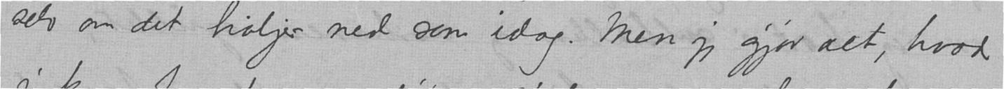selv om det høljer ned som idag. Men jeg gjør alt, hvad
